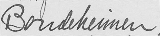Bondeheimen
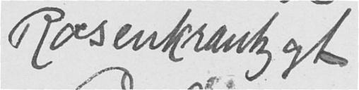Rosenkrantzgt.
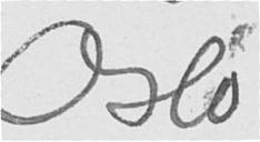Oslo
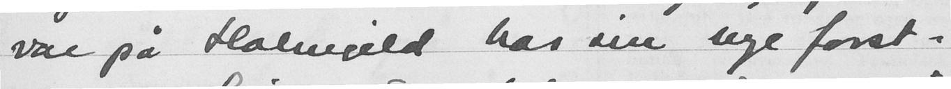var på Holmgild hos sin nye forst-
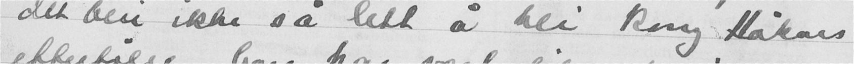det blir ikke så lett å bli kong Håkons
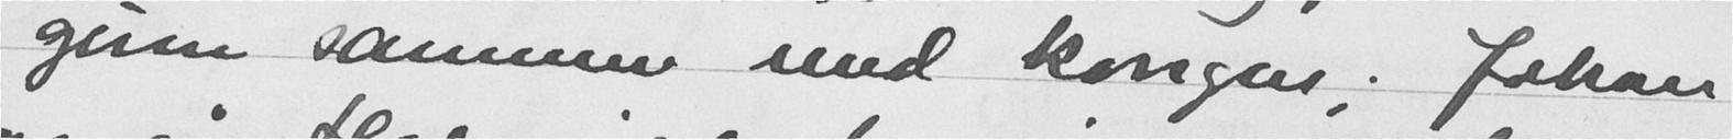gum sammn med kongen; Johan
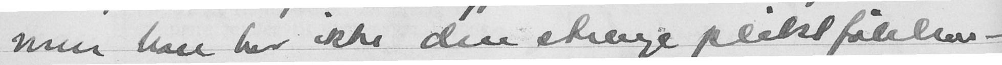men han har ikke den strenge pliktfølelsen -
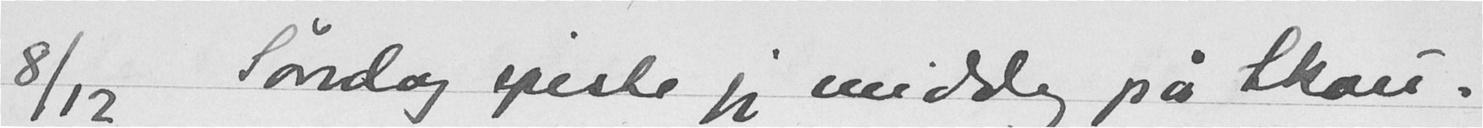8/12 Søndag spiste jeg middag på Skau-
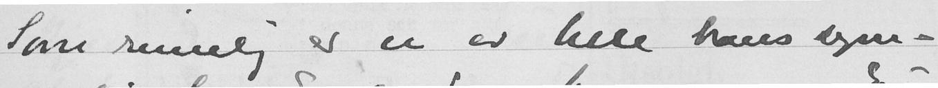Som rimelig er er er hele hans sym-
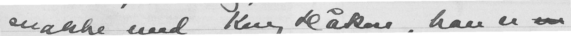snakke med Kong Håkon, han er en
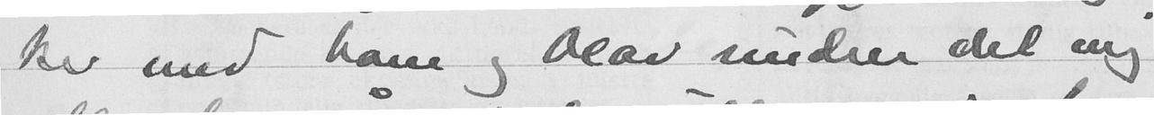er med ham og Olav undrer det mig
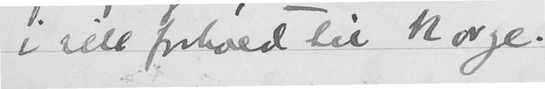i sitt forhold til Norge.
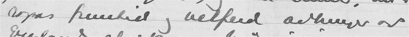ropas fremtid og velferd avhenger av
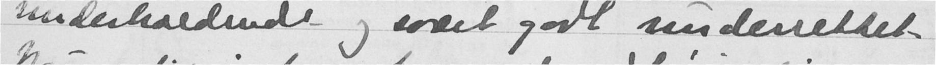underholdende og svært godt underrettet.
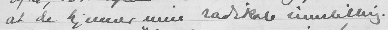at de kjenner min radikale innstilling.
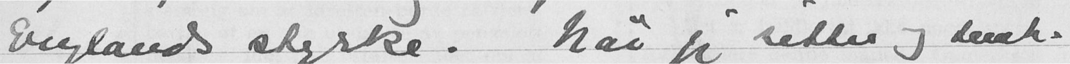Englands styrke. Når jeg sitter og tenk-
